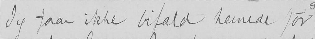Jeg faar ikke bifald hernede for
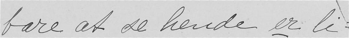bare at se hende er li-
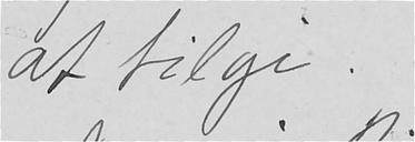at tilgi.
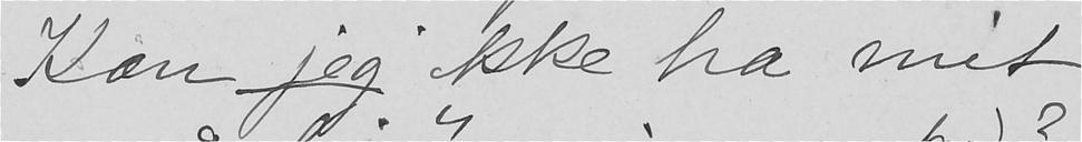Kan jeg ikke ha mit
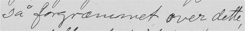så forgræmmet over dette,
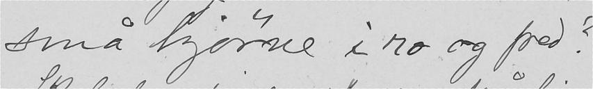små hjørne i ro og fred?
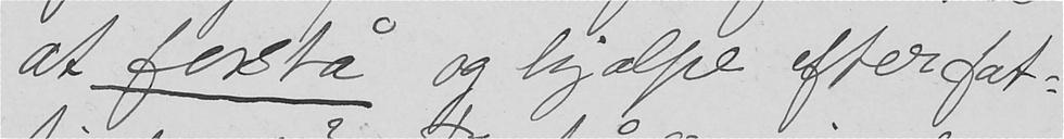at forstå og hjælpe efter fat-
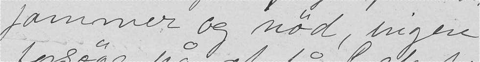jammer og nød, ingen
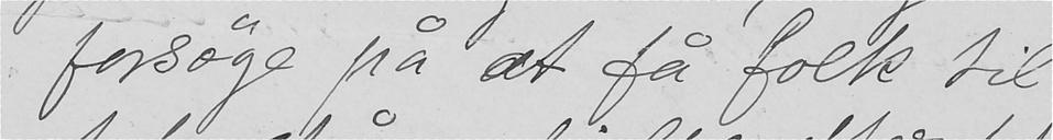forsøge på at få folk til
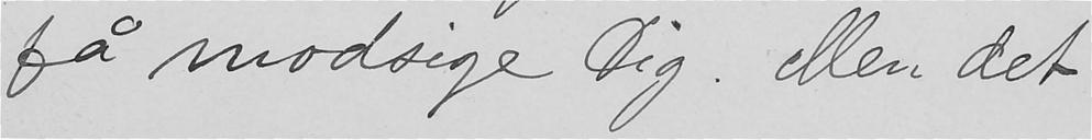få modsige Dig. Men det
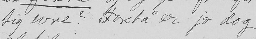tig evne? Forstå er jo dog
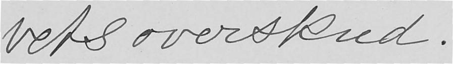vets overskud.
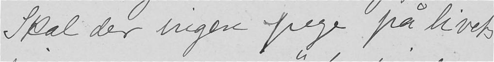Skal der ingen pege på livet
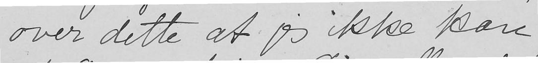over dette at jeg ikke kan
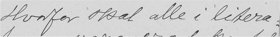Hvorfor skal alle i litera-
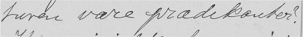turen være prædekænter?
